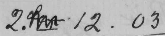2.12.03
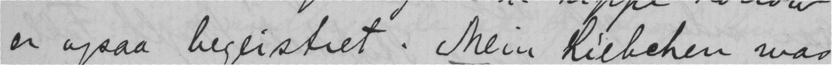er ogsaa begeistret. Mei Liebchen was
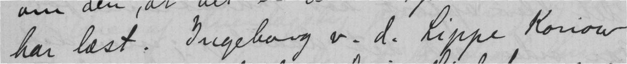har læst. Ingeborg v. d. Lippe Konow
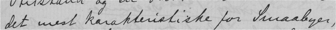det mest karakteristiske for Smaabyer,
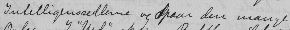Intelligenssedlerne og Spaar den mange
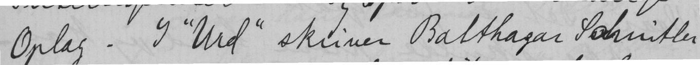Oplag. I "Urd" skriver Balthazar Schnitler
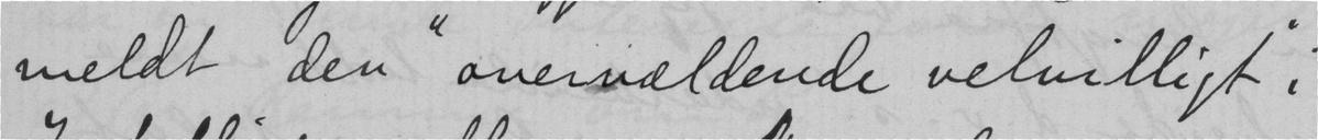meldt den "overvældende velvilligt" i
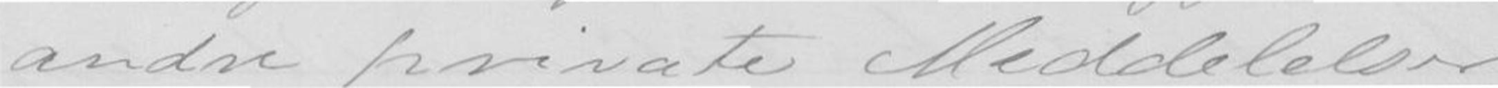andre private Meddelelser
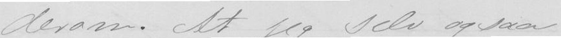derom. At jeg selv ogsaa
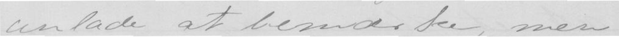unlade at bemærke, men
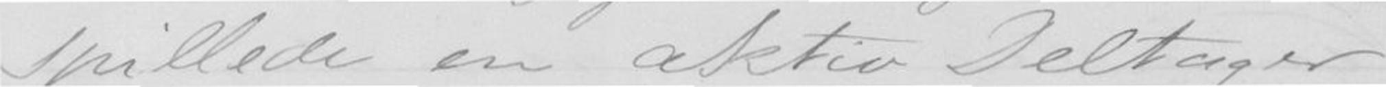spillede en aktiv Deltager
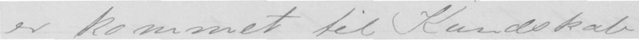er kommet til Kundskab
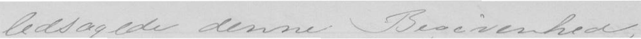ledsagede denne Begivenhed,
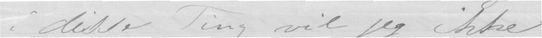i disse Ting vil jeg ikke
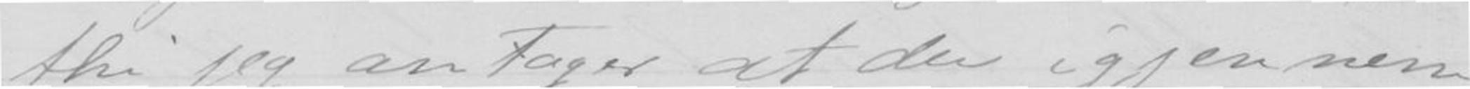thi jeg antager at du igjennem
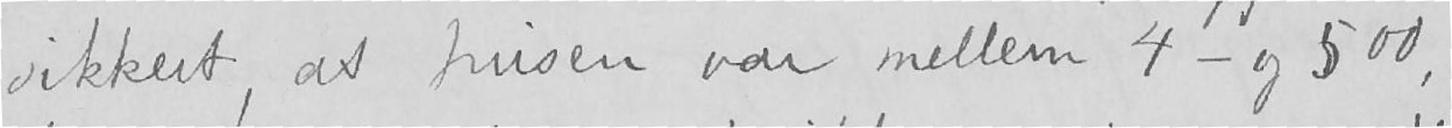sikkert, at prisen var mellem 4- og 500,
